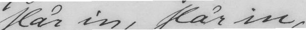slår in, slår in,
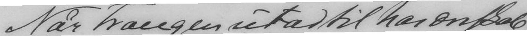Når trangen utad til har onskab,
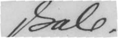skab.
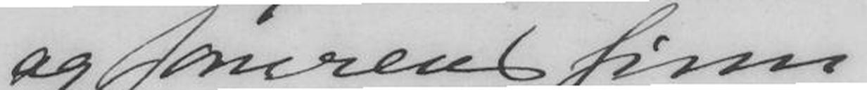og somrens sinn
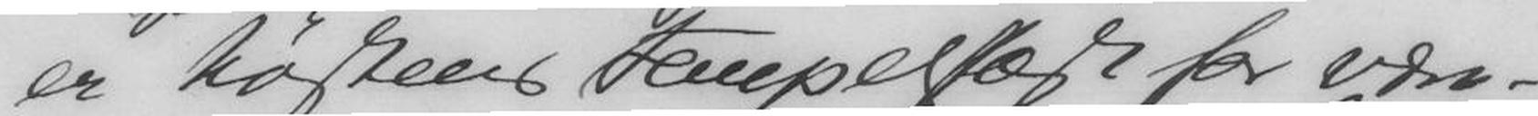er høstens tempelsfæst for væn-
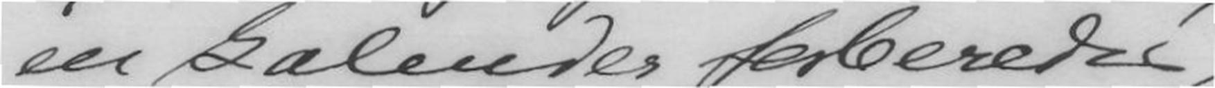en kalender forberedes,
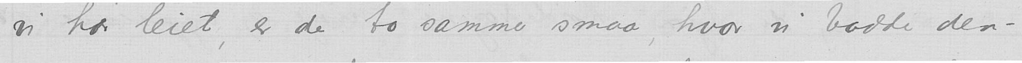vi har leiet, er de to samme smaa, hvor vi bodde den-
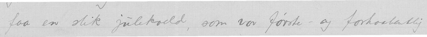faa en slik julekveld, som vor første - og forhaabentlig
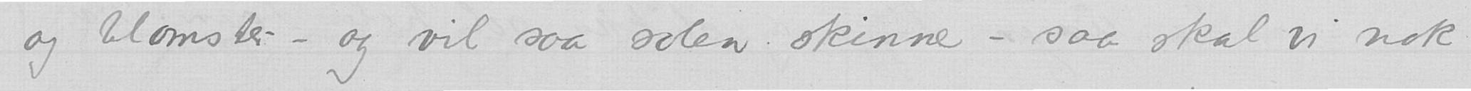og blomster - og vil saa solen skinne - saa skal vi nok
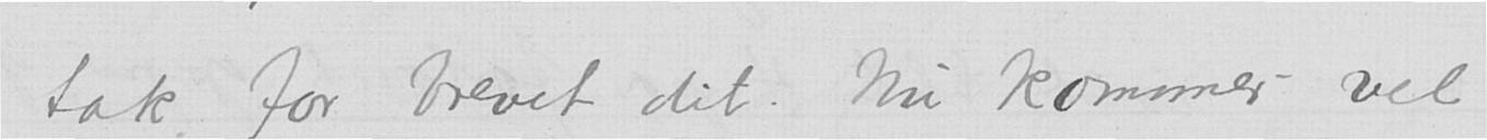tak for brevet dit. Nu kommer vel
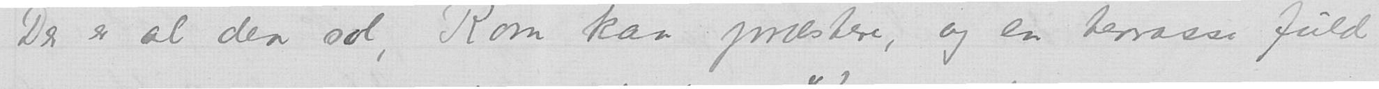Der er al den sol, Rom kan præstere, og en terrasse fuld
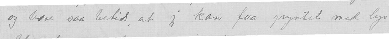- og bare saa betids, at jeg kan faa pyntet med lys
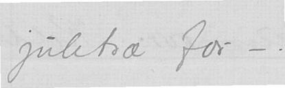juletræ for -.
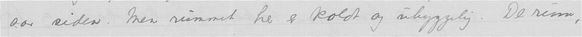aar siden. Men rummet her er koldt og uhyggelig. De rum,
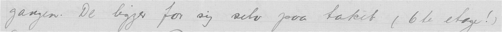gangen. De ligger for sig selv paa taket (6te etage!)
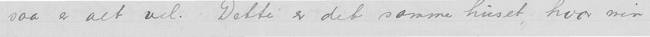saa er alt vel. Dette er det samme huset, hvor min
